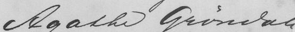Agathe Grøndahl
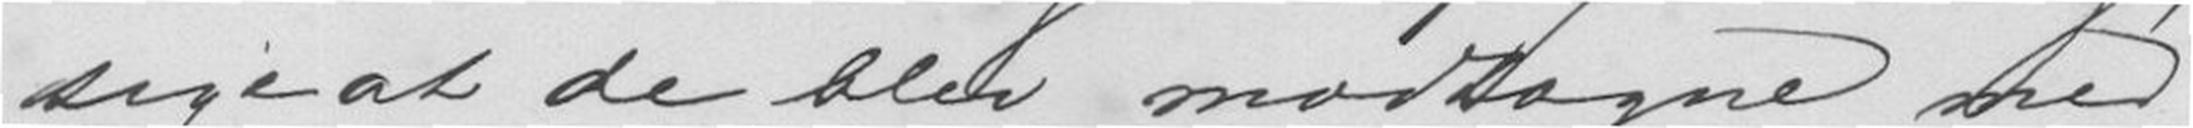sige at de blev modtagne med
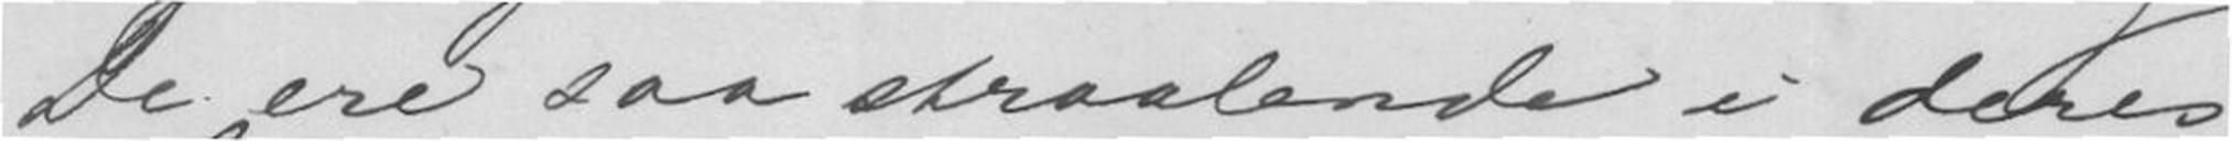De ere saa straalende i deres
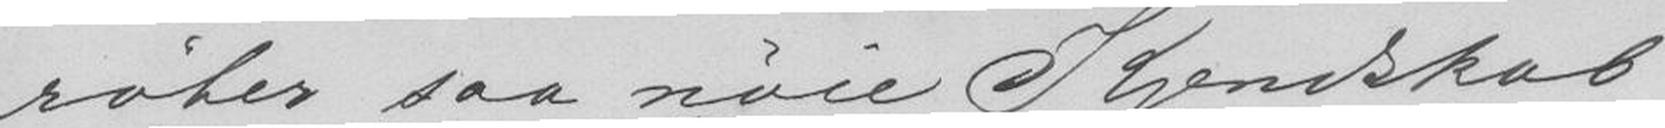røber saa nøie Kjenskab
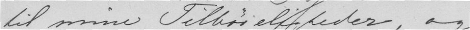til mine Tilbøieligheder, og
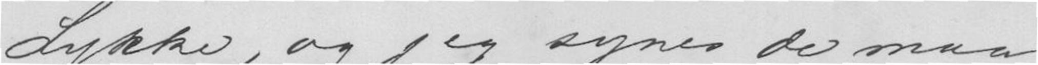Lykke, og jeg synes de maa
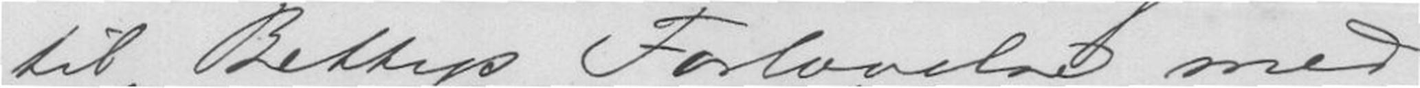til Bettys Forlovelse med
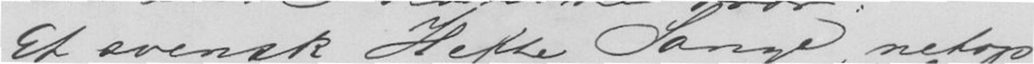Et svensk Hefte Sange, netop
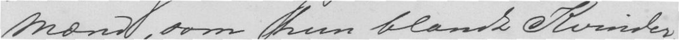Mænd, som hun blandt Kvinder,
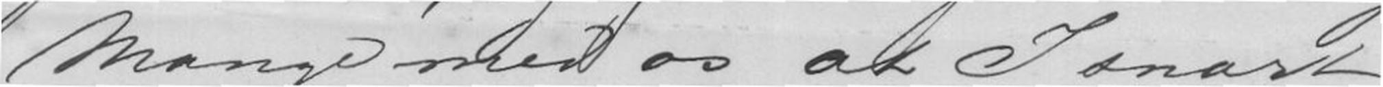Mange med os at I snart
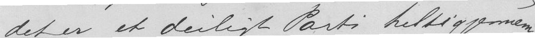det er et deiligt Parti heltigjennom.
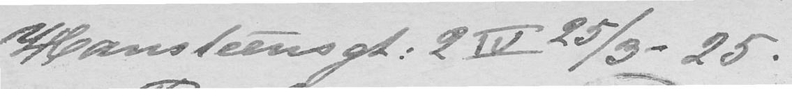Hansteensgt. 2IV 25/3 - 25.
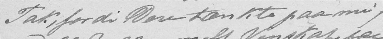Tak, fordi Dere tænkte paa mig
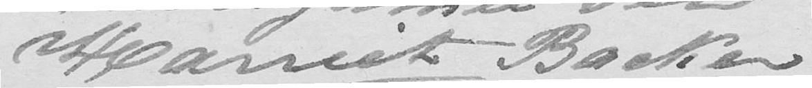Harriet Backer
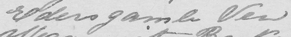Eders gamle Ven
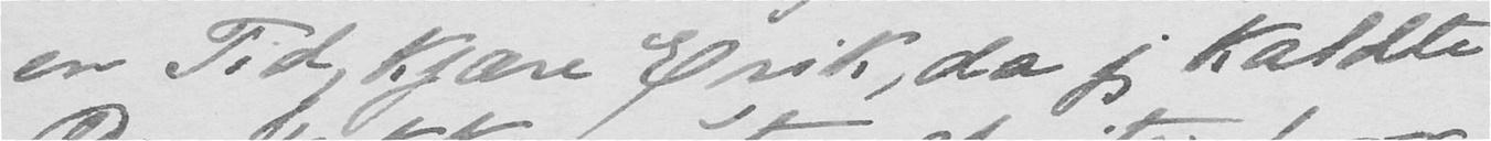en Tid, Kjære Erik, da jeg kaldte
